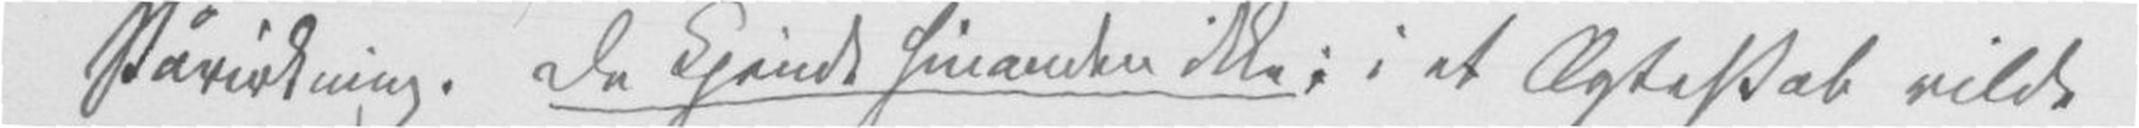Påvirkning. De kjende hinanden ikke; i et Ægteskab vilde
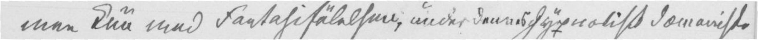men kun med Fantasifølelsen, under dennes hypnotisk dæmoniske
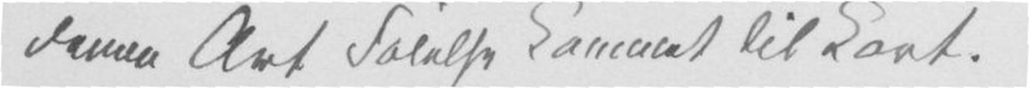denne Art Følelse kommet til Kort.
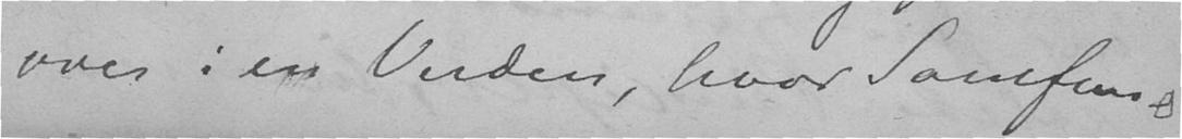over i en Verden, hvor Samfun-
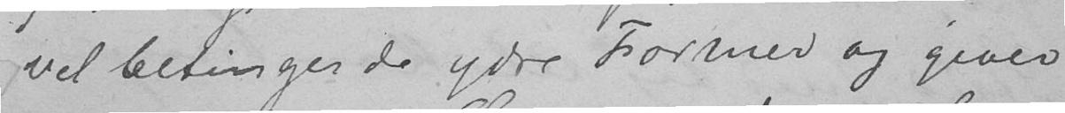vel betinger de ydre Former og giver
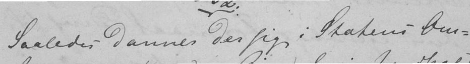Saaledes dannes der sig i Statens Om-
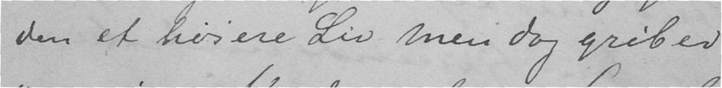den et høiere Liv, men dog griber
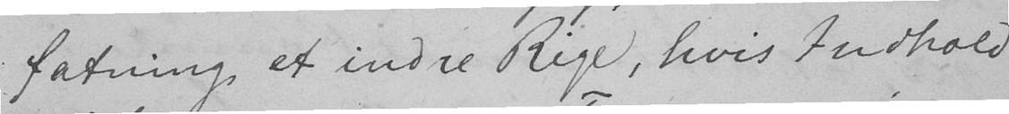fatning et indre Rige, hvis Indhold
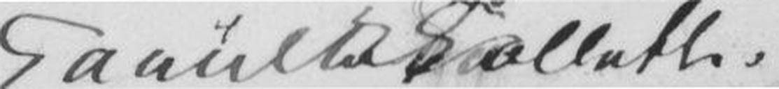Camilla Collett.
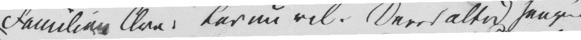Familien Ære. Far nu vel. Deres altid hengivne
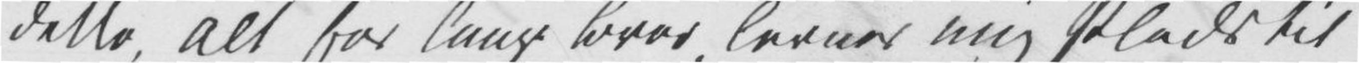i dette, alt for lange Brev, levnes mig Plads til
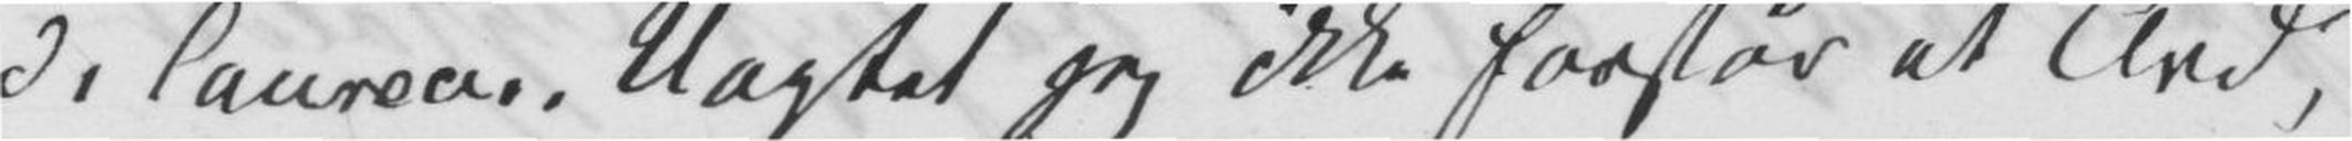di Laurea. Uagtet jeg ikke forstår et Ord,
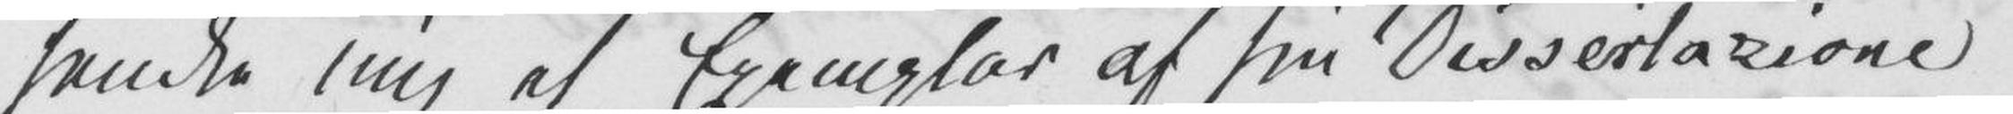sendte mig et Exemplar af sin Dissertazione
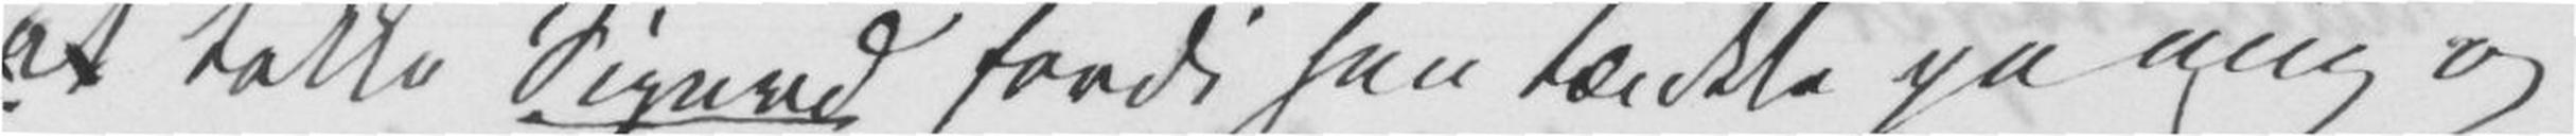at takke Sigurd fordi han tænkte på mig og
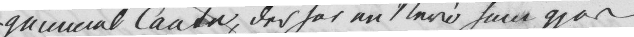gammel Tante, der har en Nevø som gjør
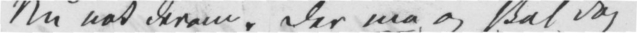Nu nok derom. Der må og skal dog
